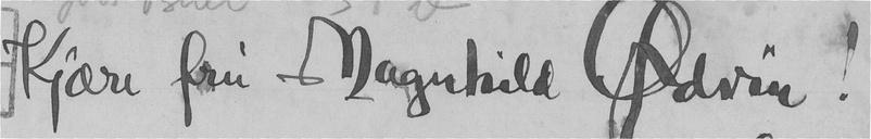Kjære fru Magnhild Ødvin!
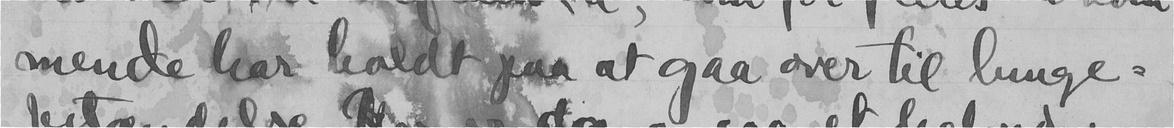mende har holdt paa at gaa over til lunge-
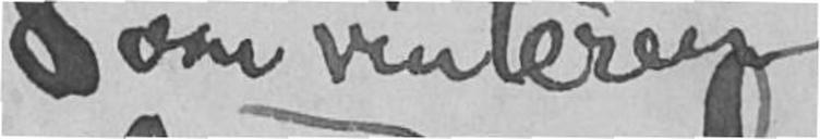om vinteren
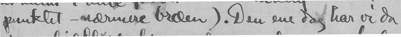punktet - nærmere bræen). Den ene dag har vi da
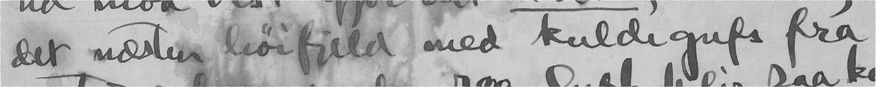det næsten høifjeld med kulde gufs fra
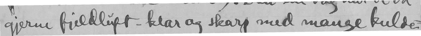gjerne fjeldluft klar og skarp med mange kulde-
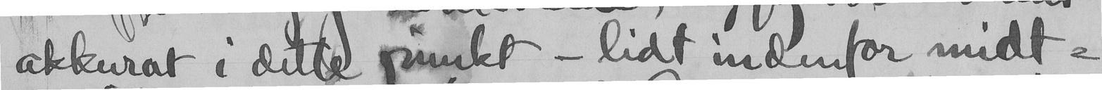akkurat i dette punkt - lidt indenfor midt-
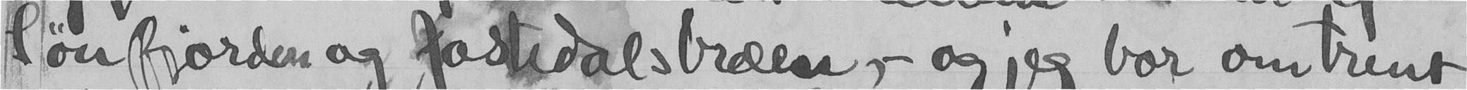Lønfjorden og Jostedalsbræen", - og jeg bor omtrent
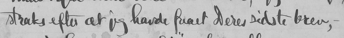strak efter at jeg havde faaet Dere sidste brev, -
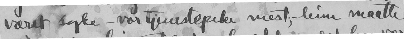været syke - vor tjenestepike mest, - hun maate
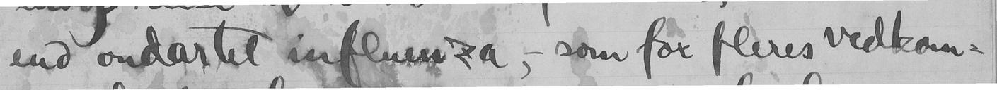end ondartet influenza, - som for fleres vedkom-
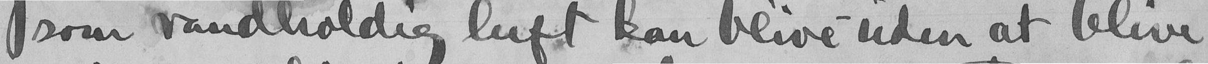som vandholdig luft kan blive uden at blive
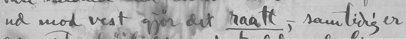ud mod vest gjør det raatt, - samtidig er
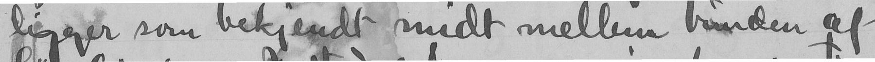ligger som bekjendt midt mellem bunden af
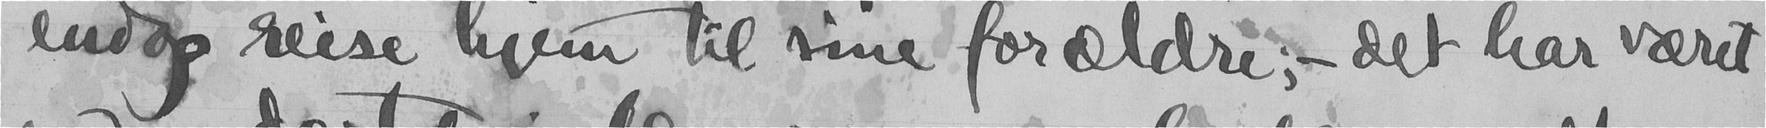endop reise hjem til sine forældre; - det har været
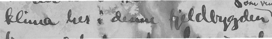klina her i denne fjeldbygden
